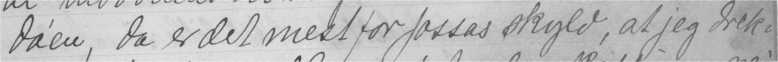da'en, da er det mest for Jossas skyld, at jeg drek-
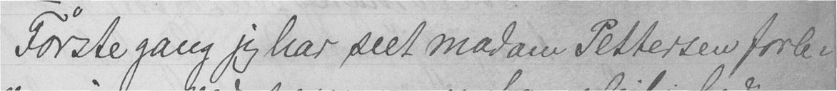Første gang jeg har seet madam Peftersen forle-
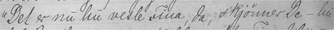"Det er nu hu vesle Tina, da, skjønner De - hu
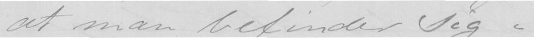at man befinder sig i
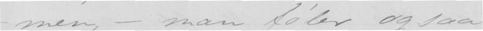- men, - man føler ogsaa
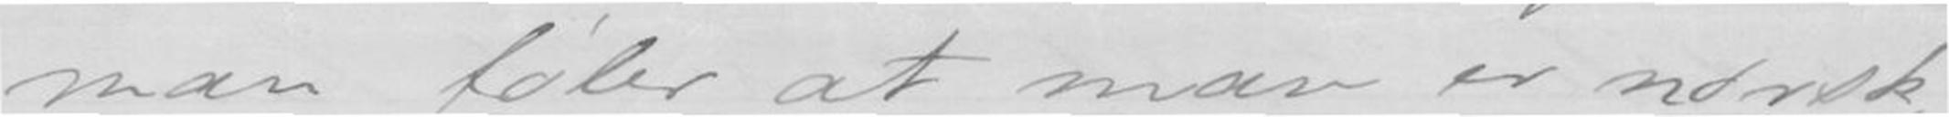man føler at man er norsk,
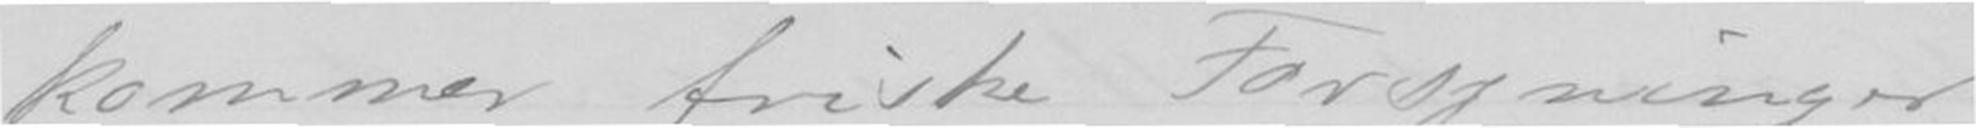kommer friske Forsyninger
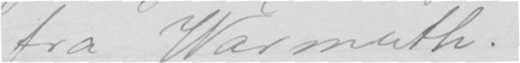fra Warmuth. -
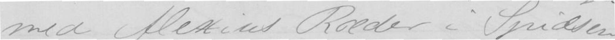med, Alexius Ræder i Spidsen
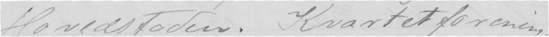Hovedstaden. Kvartetforeningen
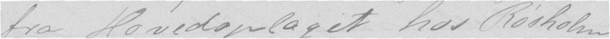fra Hovedoplaget hos Røsholm
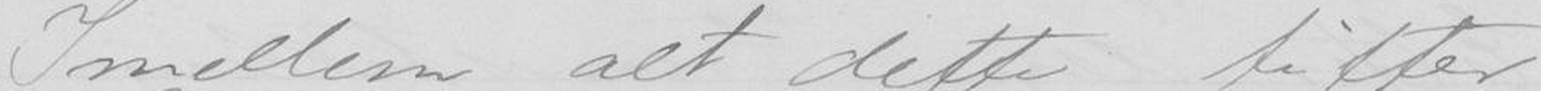I mellem alt dette titter
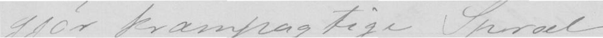gjør krampagtige Spræl
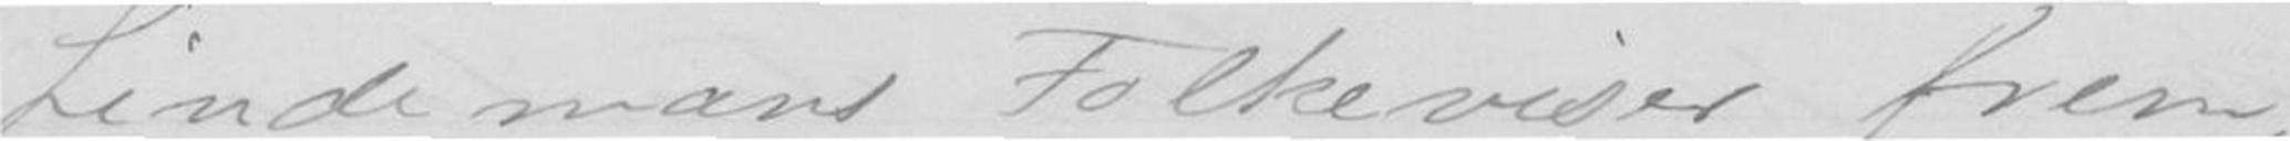Lindemans Folkeviser frem,
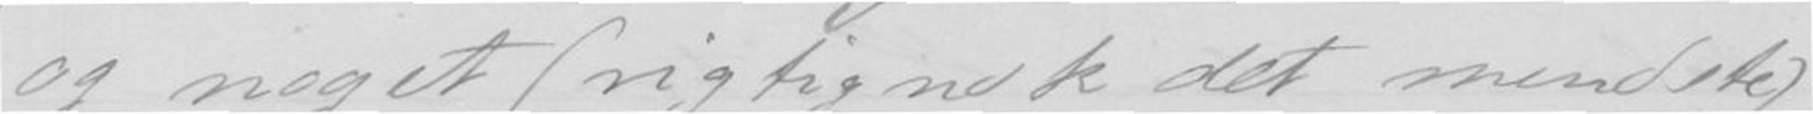og noget (rigtignok det mindste)
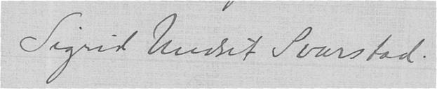Sigrid Undset Svarstad.
